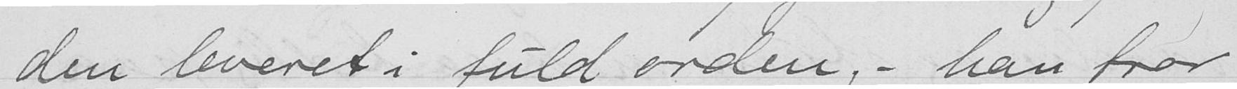den leveret i fuld orden, - han tror
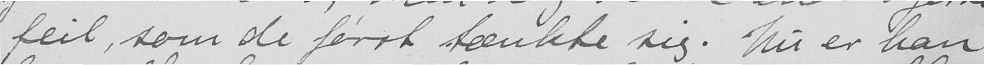feil, som de først tænkte sig. Nu er han
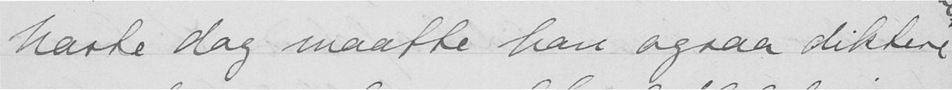Næste dag maatte han ogsaa diktere
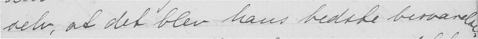selv, at det blev hans bedste besvarelse.
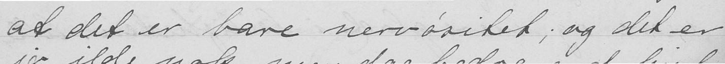at det er bare nervøsitet; og det er
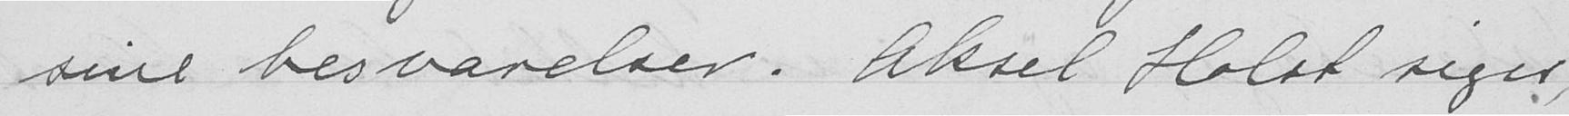sine besvarelser. Aksel Holst siger,
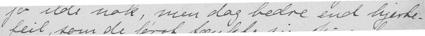jo ilde nok, men dog bedre end hjerte-
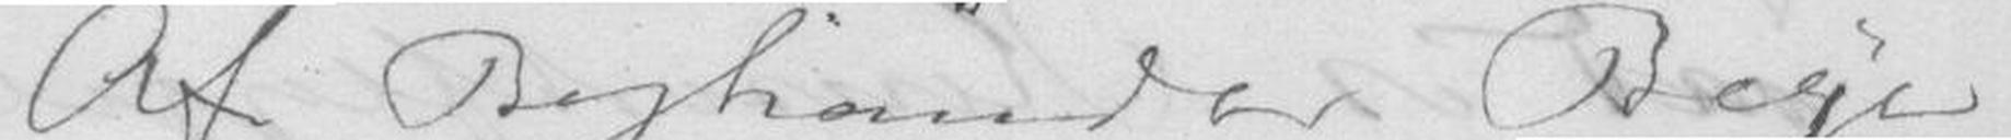Af Boghandler Beyer
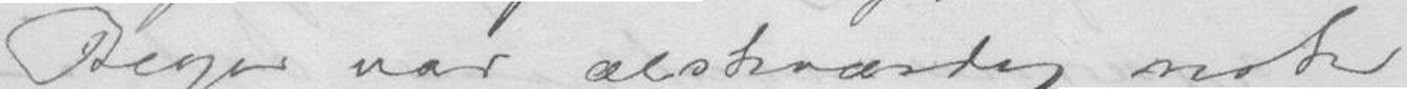Beyer var ælskværdig nok
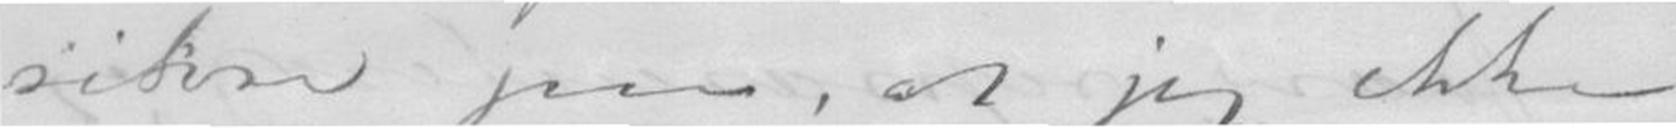sikre paa, at jeg ikke
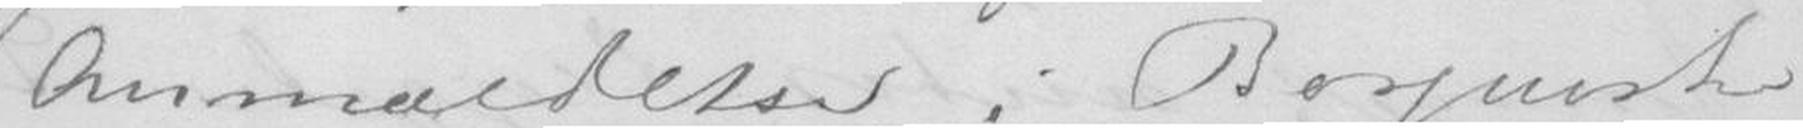Anmældelse i Bergenske
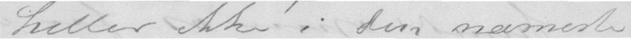heller ikke i den nærmeste
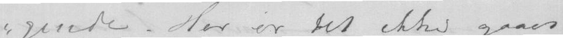gende. Her er det ikke gaaet
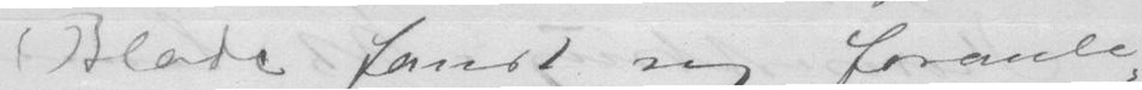Blade fandt sig foranle-
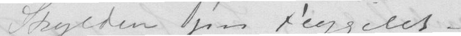Skylden paa Flygelet -
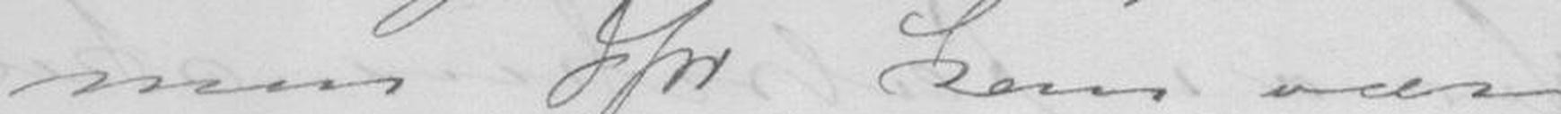men Dfor kan være
